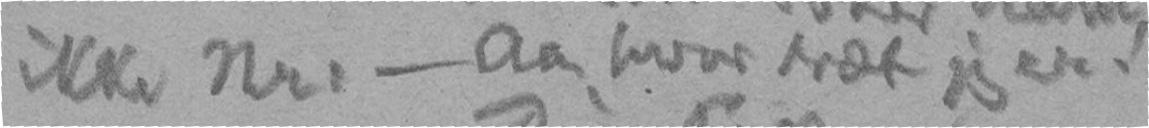ikke Nr. - Aa hvor træt jeg er!
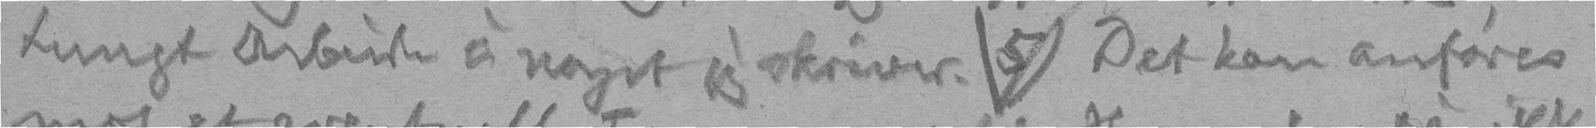tungt Arbeide i noget jeg skriver. 5) Det kan anføres
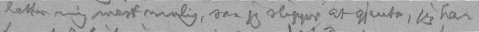letter mig mest mulig, saa jeg slipper at gjenta, jeg har
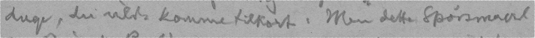duge, du vilde komme tilkort. Men dette Spørsmaal
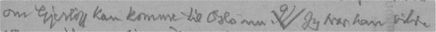om Gierløff kan komme til Oslo nu. 9) Jeg tror han vilde
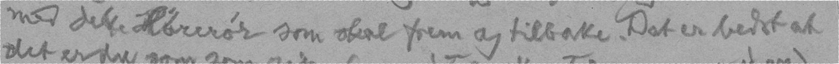med dette Hørerør som skal frem og tilbake. Det er bedst at
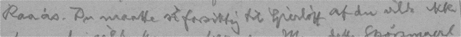Raa'as. Du maatte si forsiktig til Gierløff at du vilde ikke
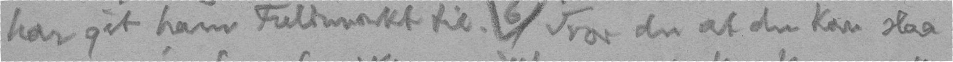har git ham Fuldmakt til. 6) Tror du at du kan slaa
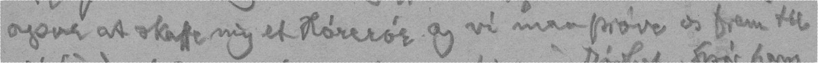ogsaa at skaffe mig et Hørerør og vi maa prøve os frem til
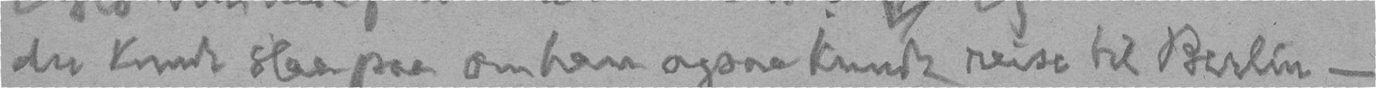du kunde slaa paa om han ogsaa kunde reise til Berlin -
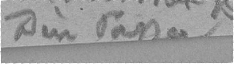Din Papa
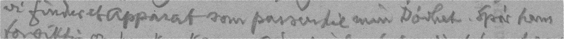vi finder et Apparat som passer til min Døvhet. Spør ham
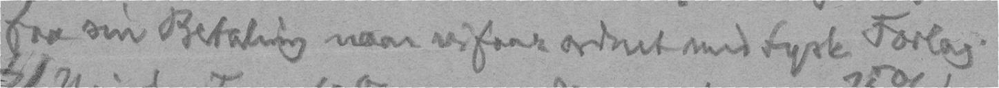faa sin Betaling naar vi faar ordnet med tysk Forlag.
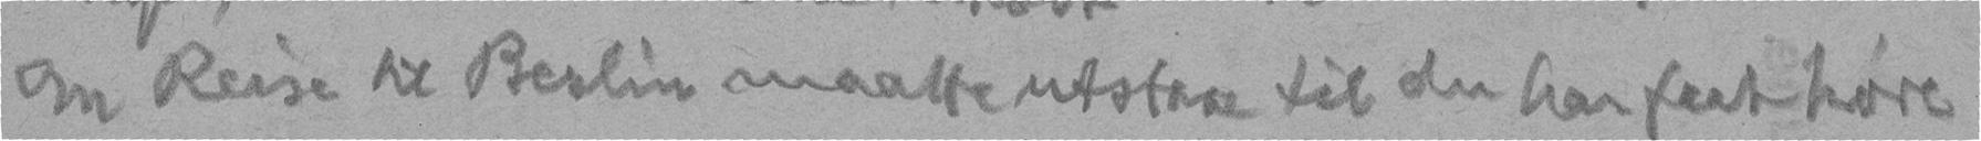om Reise til Berlin maatte utstaa til du har faat høre
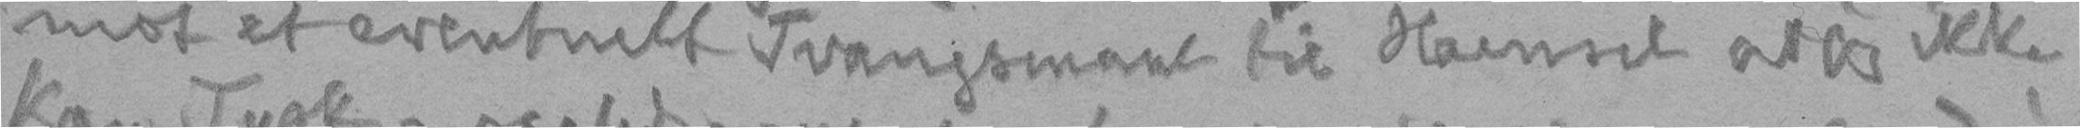mot et eventuelt Tvangsmaal til Haensel at jeg ikke
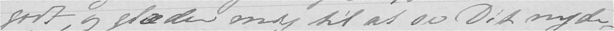godt, og glæder mig til at se Dit nyde-
